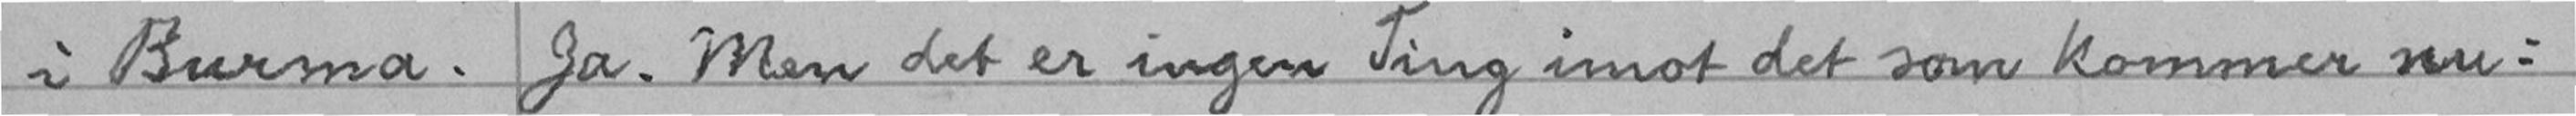i Burma. Ja. Men det er ingen Ting imot det som kommer nu:
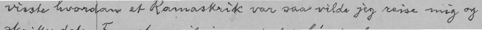visste hvordan et Ramaskrik var saa vilde jeg reise mig og
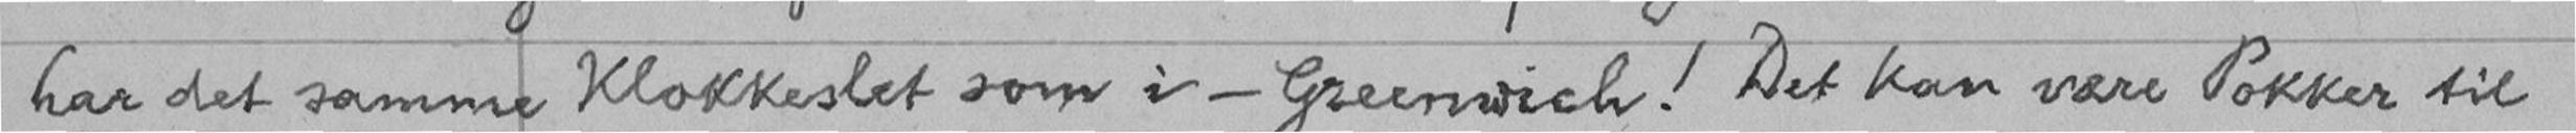har det samme Klokkeslet som i - Greenwich! Det kan være Pokker til
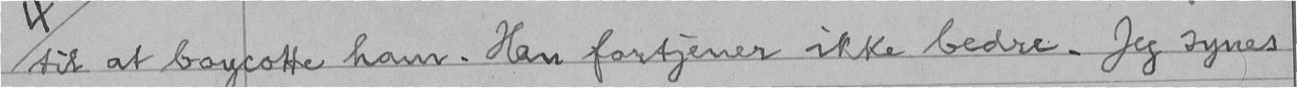til at boycotte ham. Han fortjener ikke bedre. Jeg synes
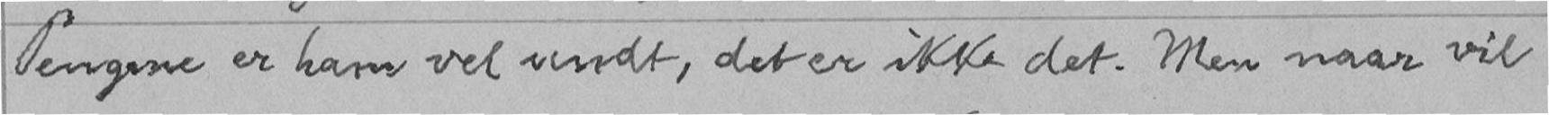Pengene er ham vel undt, det er ikke det. Men naar vil
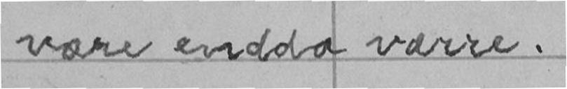være endda værre.
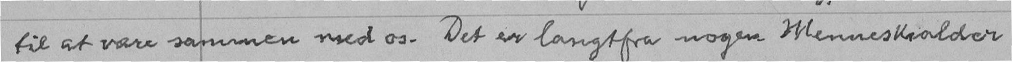til at være sammen med os. Det er langtfra nogen Menneskealder
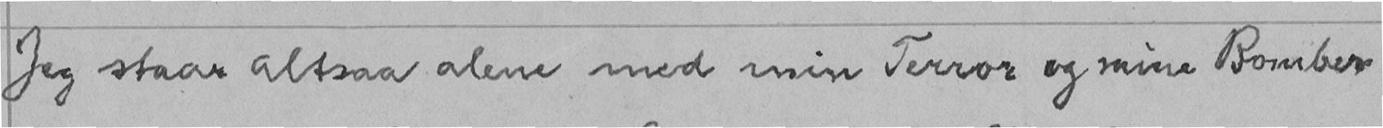Jeg staar altsaa alene med min Terror og mine Bomber
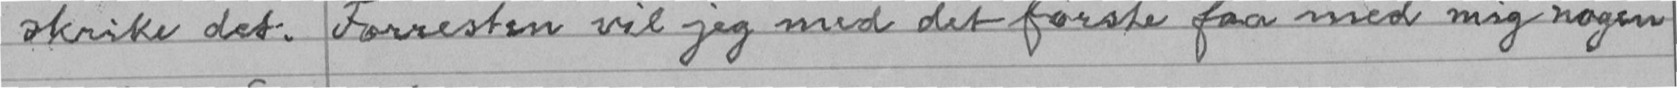skrike det. Forresten vil jeg med det første faa med mig nogen
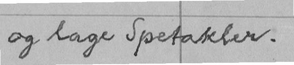og lage Spetakler.
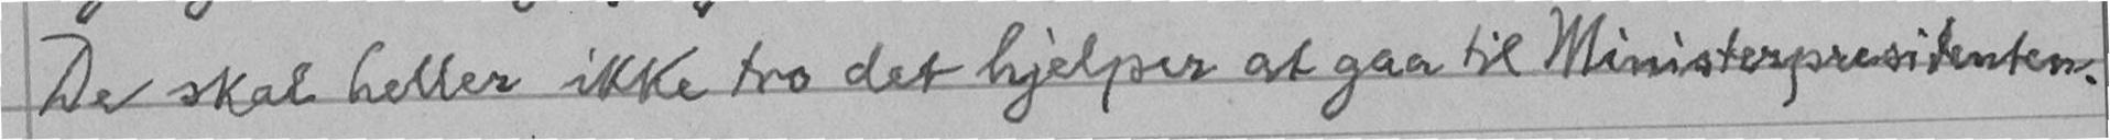De skal heller ikke tro det hjelper at gaa til Ministerpresidenten.
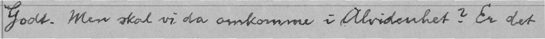Godt. Men skal vi da omkomme i Alvidenhet? Er det
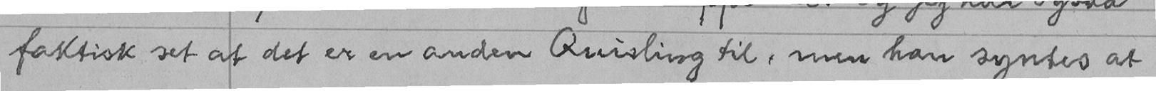faktisk set at det er en anden Quisling til, men han syntes at
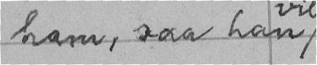ham, saa han
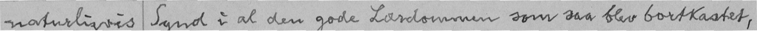naturligvis Synd i al den gode Lærdommen som saa blev bortkastet,
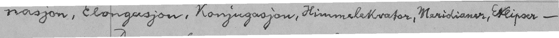nasjon, Elongasjon, Konjugasjon, Himmelekvator, Meridianen, Eklipser -
